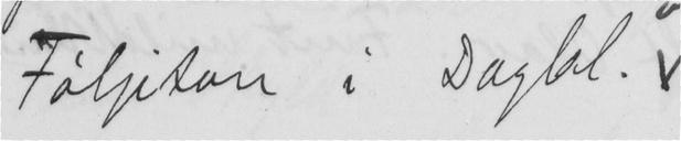Føljeton i Dagbl.
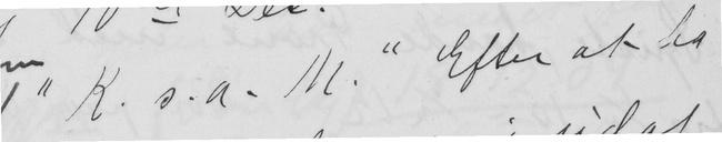"K. s. a. M." Efter at ha
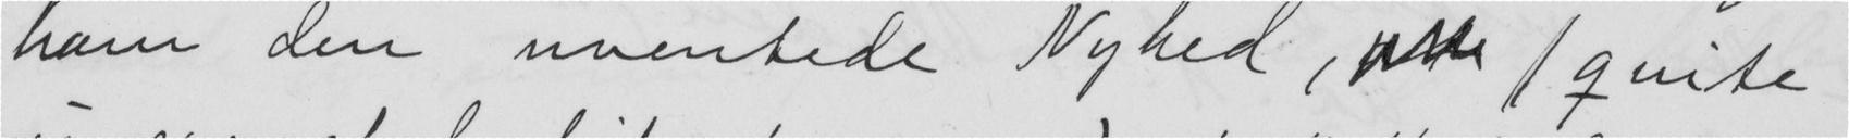ham den uventede Nyhed,   (quite
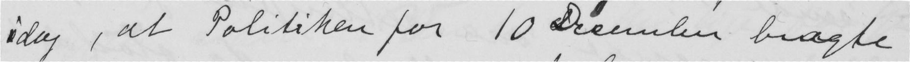idag, at Politiken for 10 December bragte
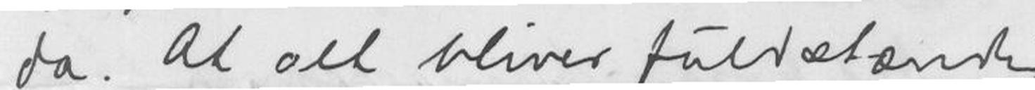da. At alt bliver fuldstændig
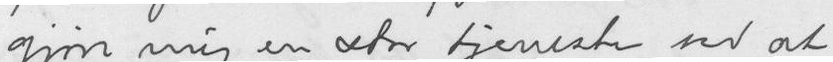gjøre mig en stor tjeneste ved at
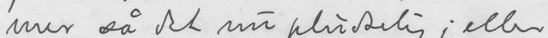mer så det nu pludselig, eller
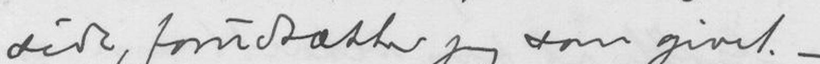side, forudsætter jeg som givet.-
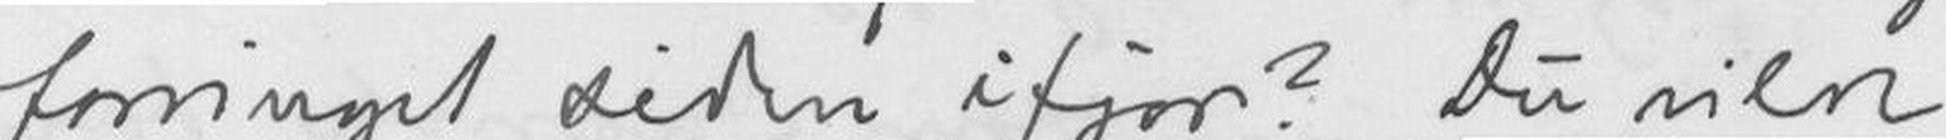for ringet siden ifjor? Du vilde
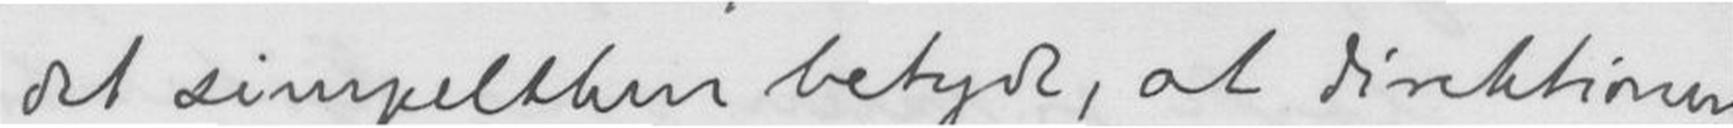det simpelthen betyde, at direktionen
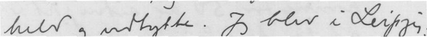held og udbytte. Jeg blev i Leipzig;
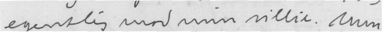egentlig mod min villie. Men
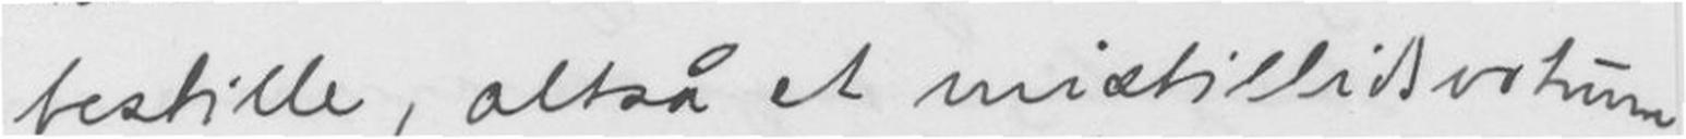bestille, altså et mistillidsvotum
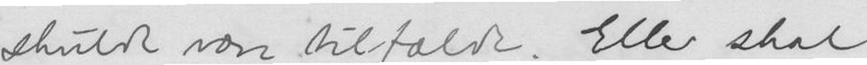skulde være tilfælde. Eller skal
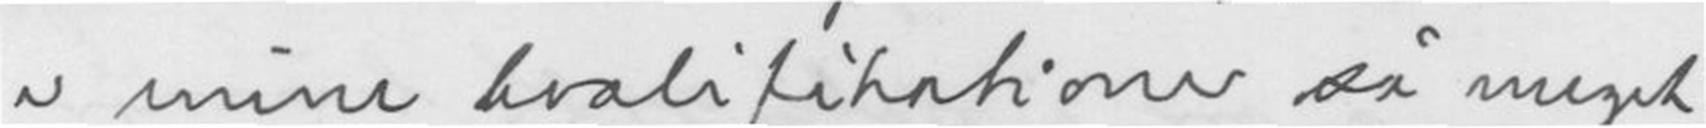er mine kvalifikationer så meget
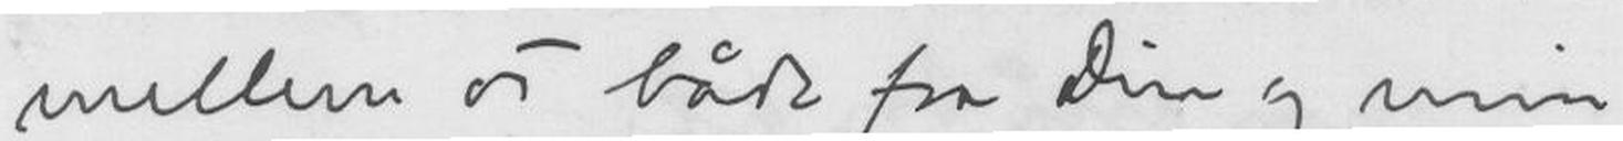mellem os både fra Din og min
